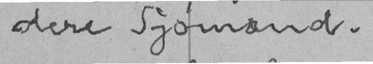dere Sjømænd.
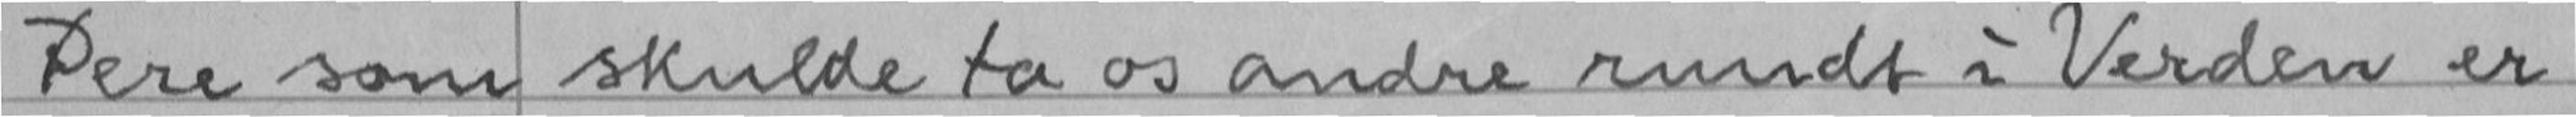Dere som skulde ta os andre rundt i Verden er
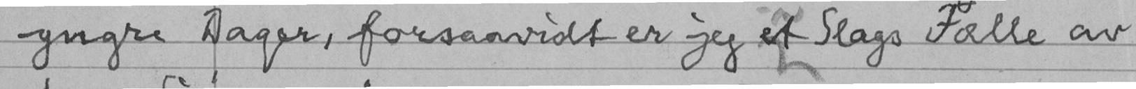yngre Dager, forsaavidt er jeg et Slags Fælle av
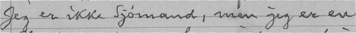Jeg er ikke Sjømand, men jeg er en
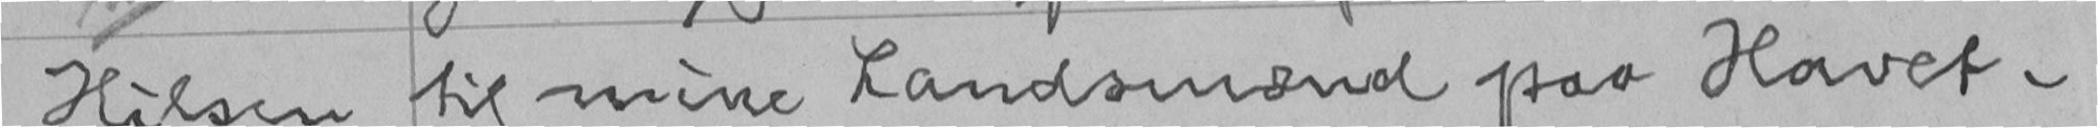Hilsen til mine Landsmænd paa Havet.
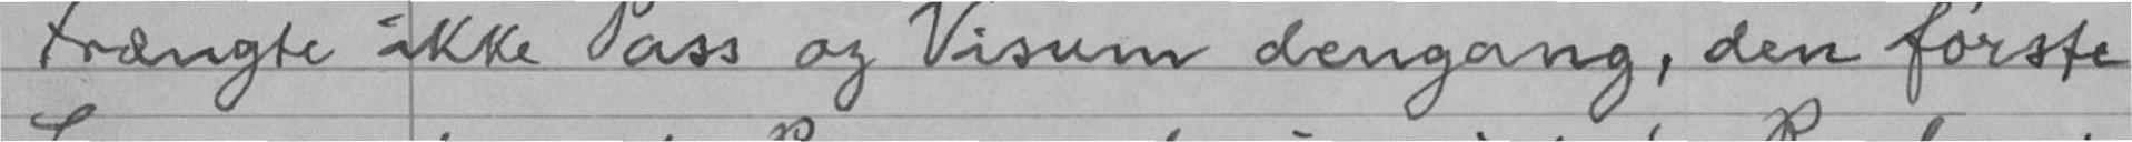trængte ikke Pass og Visum dengang, den første
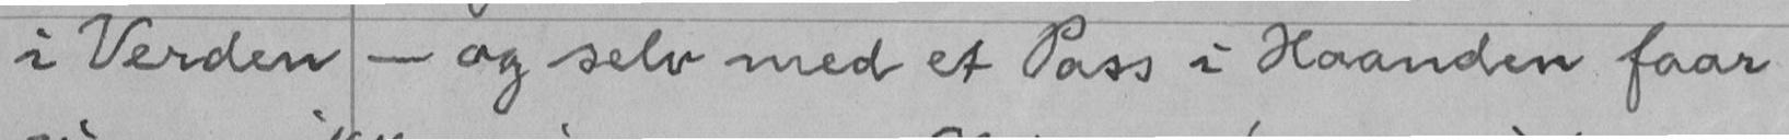i Verden - og selv med et Pass i Haanden faar
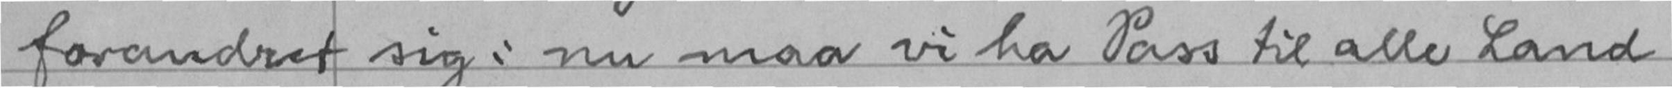forandret sig: nu maa vi ha Pass til alle Land
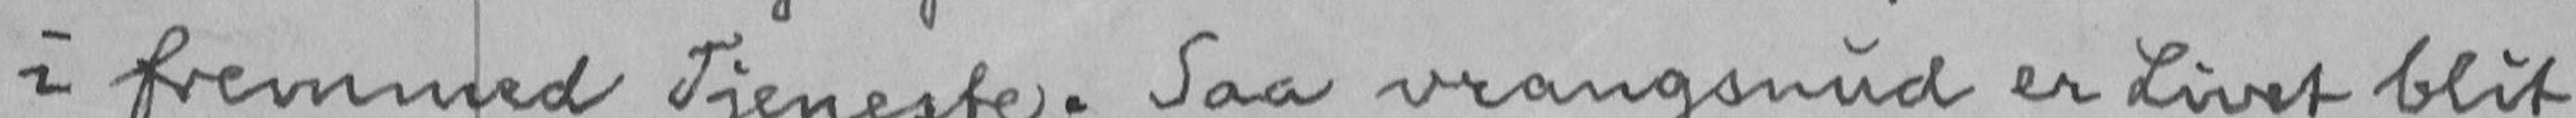i fremmed Tjeneste. Saa vrangsnud er Livet blit
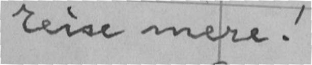reise mere!
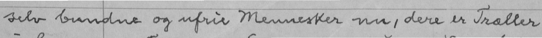selv bundne og ufrie Mennesker nu, dere er Træller
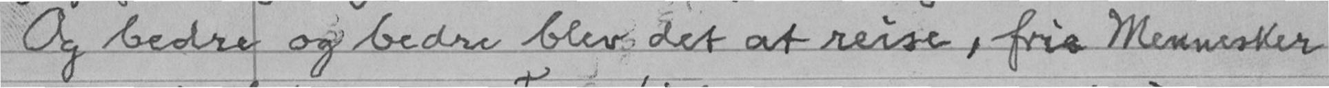Og bedre og bedre blev det at reise, frie Mennesker
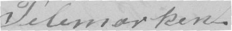Telemarken
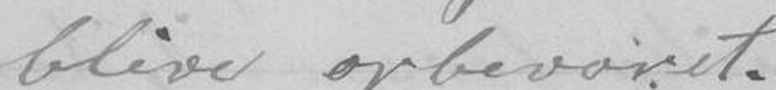blive opbevaret.
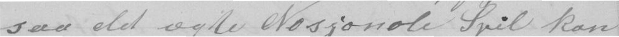saa det ægte Nasjonale Spil kan
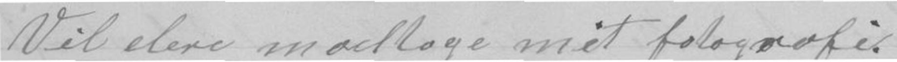Vil dere modtage mit fotografi.
